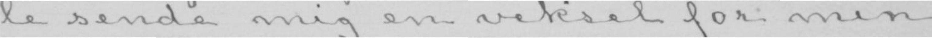le sende mig en veksel for min
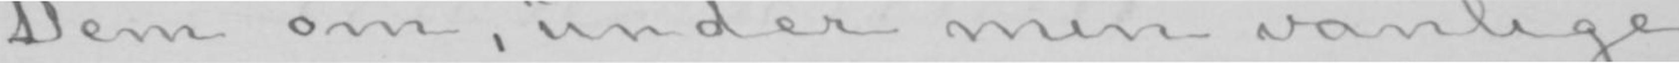Dem om, under min vanlige
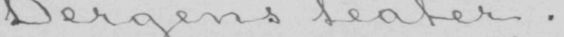Bergens teater.
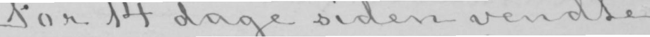For 14 dage siden vendte
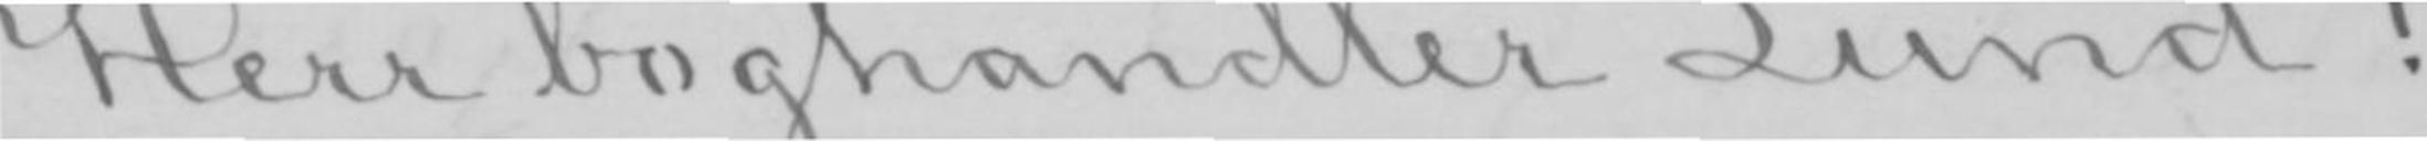Herr boghandler Lund!
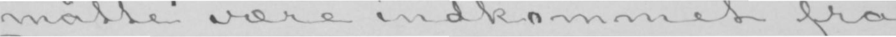måtte være indkommet fra
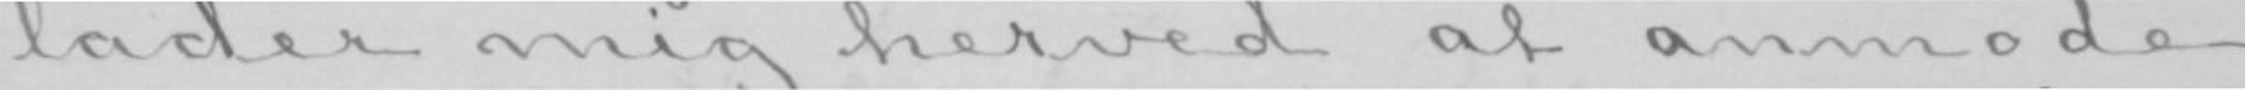lader mig herved at anmode
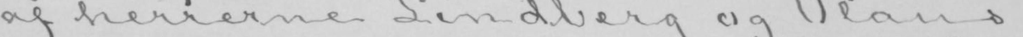af herrerne Lindberg og Olaus
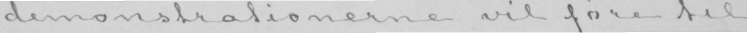demonstrationerne vil føre til
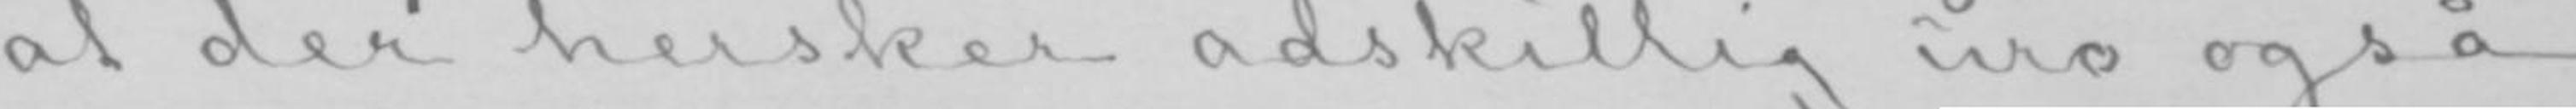at der hersker adskillig uro også
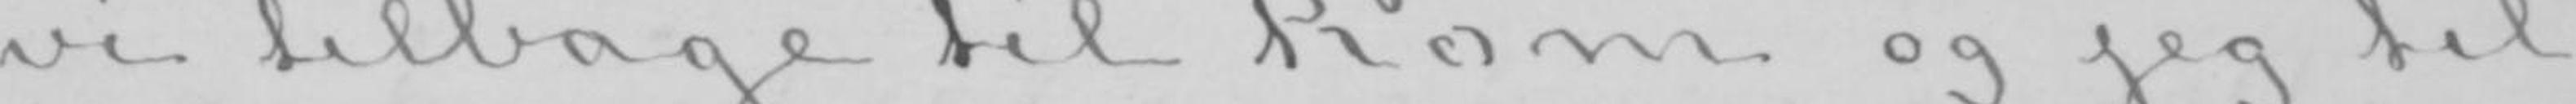vi tilbage til Rom og jeg til-
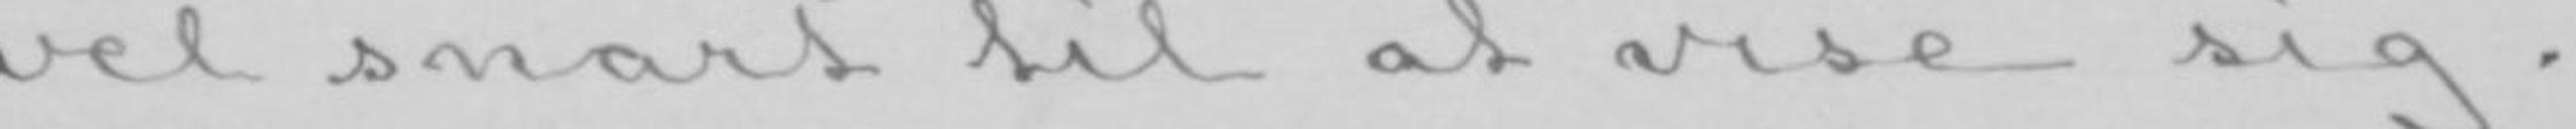vel snart til at vise sig.
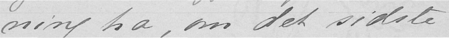ning ha, om det sidste
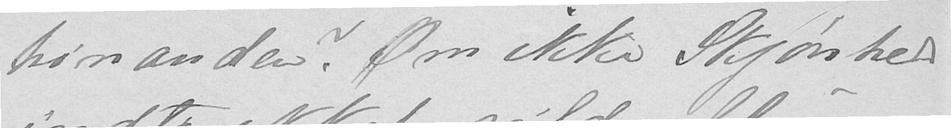hinanden? Om ikke Skjønheds-
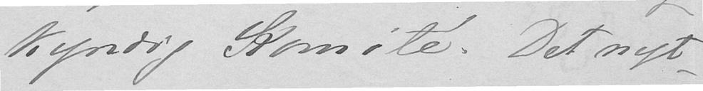kyndig Komité. Det nyt-
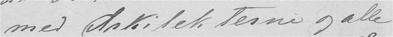med Arkitekterne og alle
